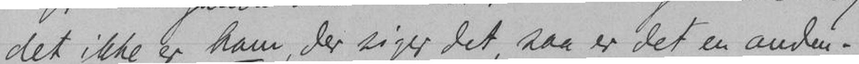det ikke er ham, der siger det, saa er det en anden.
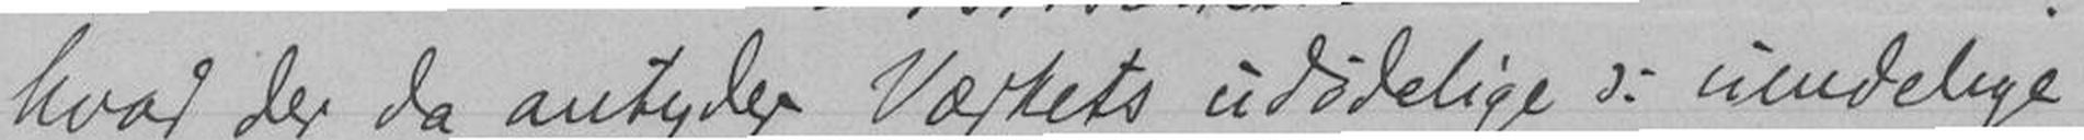hvad der da antyder Værkets udødekige d: uendelige
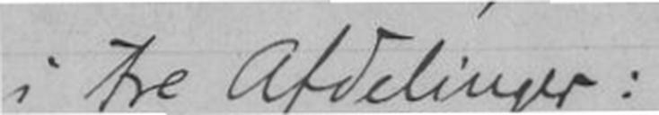i tre Afdelinger:
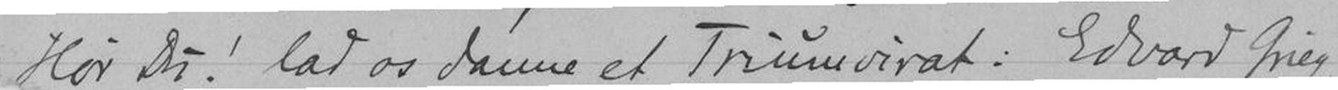næste Aar? Hør Du! lad os danne et Triumvirat: Edvard Grieg
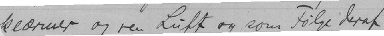klærmer og ren Luft og som Følge deraf
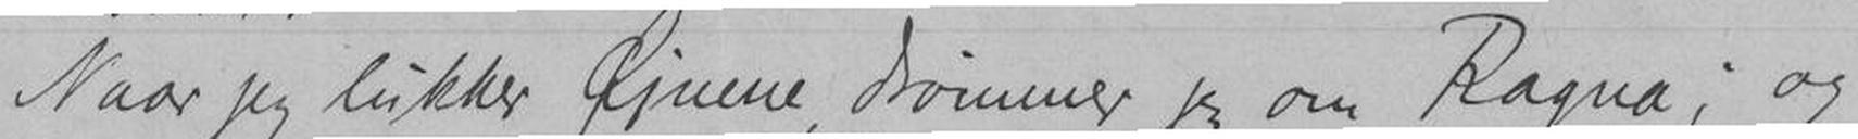Naar jeg lukker Øjnene drømmer jeg om Ragna; og
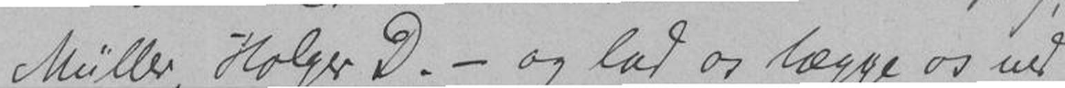Müller, Holger D. - og lad os lægge os ud
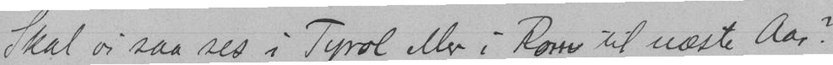Skal vi saa ses i Tyrol eller i Rom til
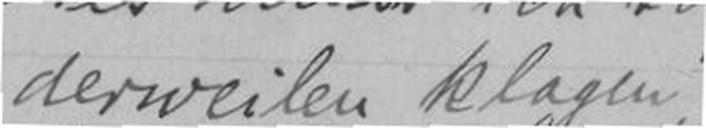derweilen klagen,
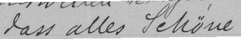dass alles Schöne
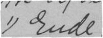1) Ende
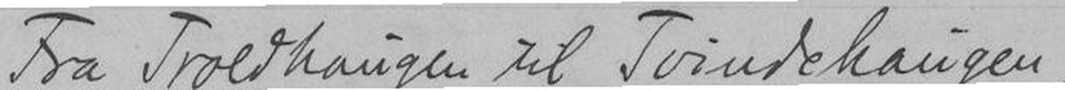Fra Troldhaugen til Tvindehaugen,
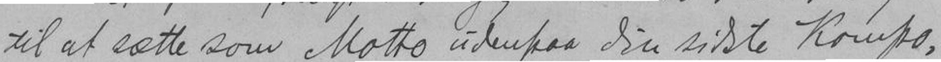til at sætter som Motto udenpaa din sidste Kompo-
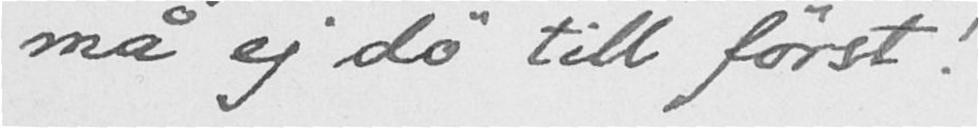må ej dö till først!
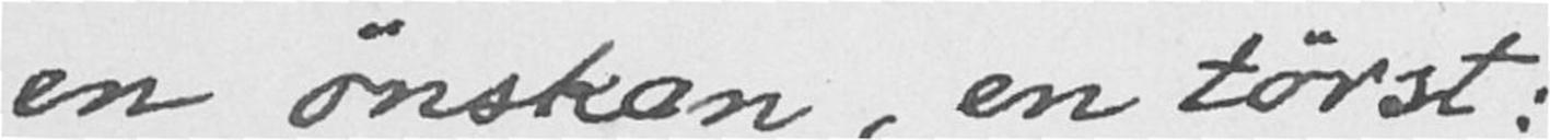en önskan, en törst:
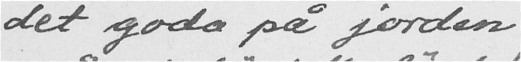det goda på jorden
